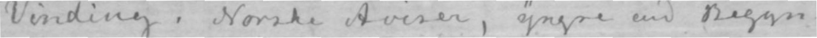Vinding. Norske Aviser, yngre end Begyn-
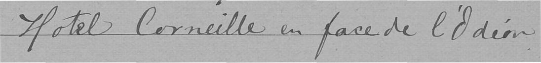Hotel Corneille en face de l'Edior
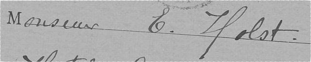Monseur E. Holst.
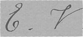E. V.
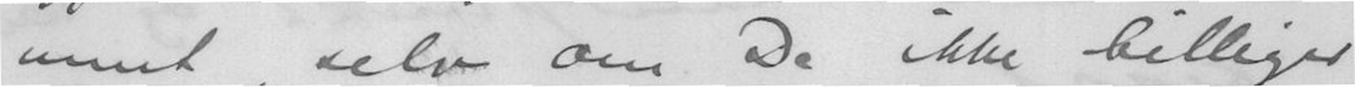ment, selv om De ikke billiger
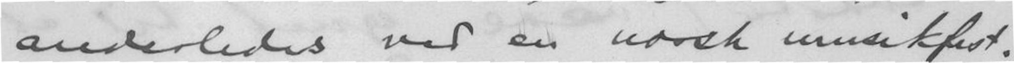anderledes ved en nosk musikfest.
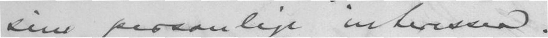sine personlige interesser.
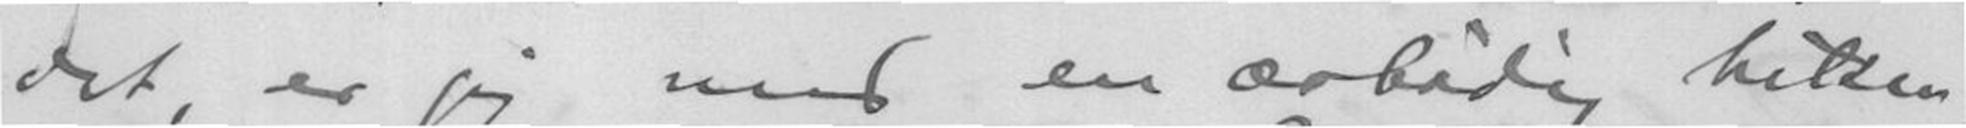det, er jeg med en ærbødig hilsen
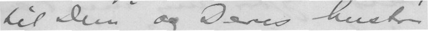til Dem og Deres hustru
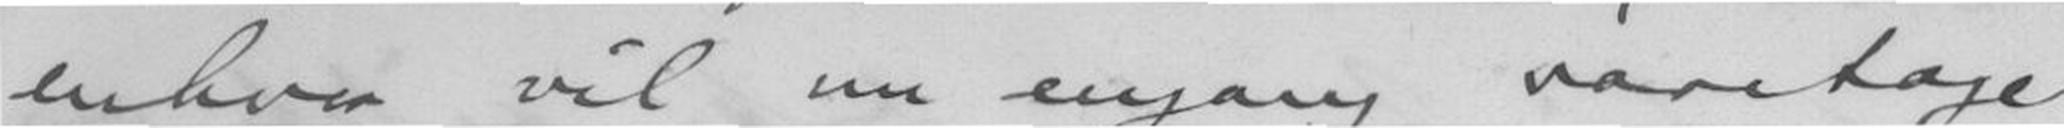enhver vil nu engang varetage
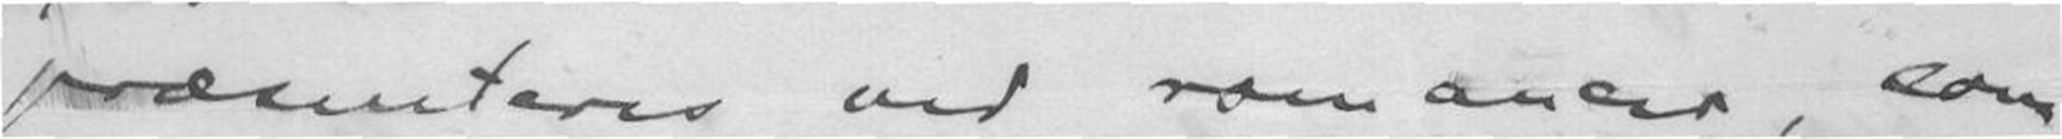præsenteres ved romancer, som
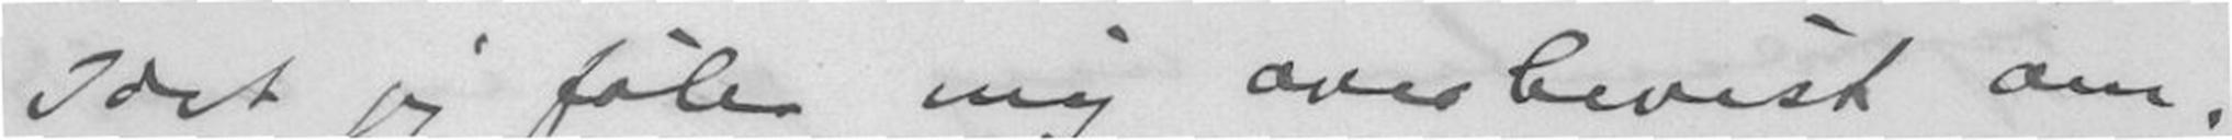Idet jeg føler mig overbevist om,
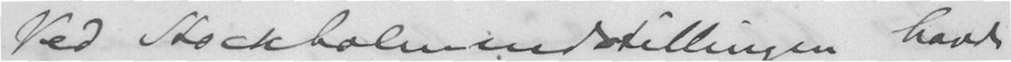Ved Stocholmsudstillingen havde
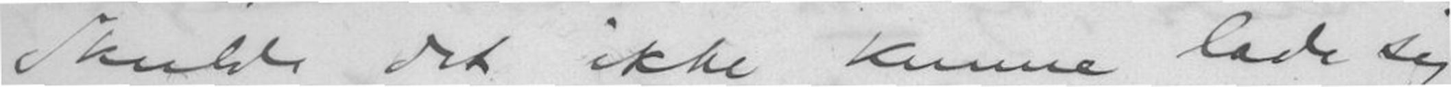Skulde det ikke kunne lade sig
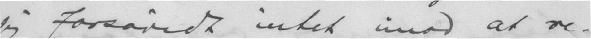jeg fosåvidt intet imod at re-
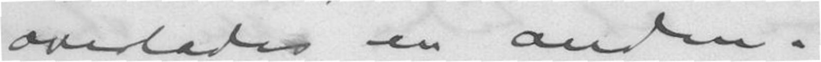overlades en anden.
